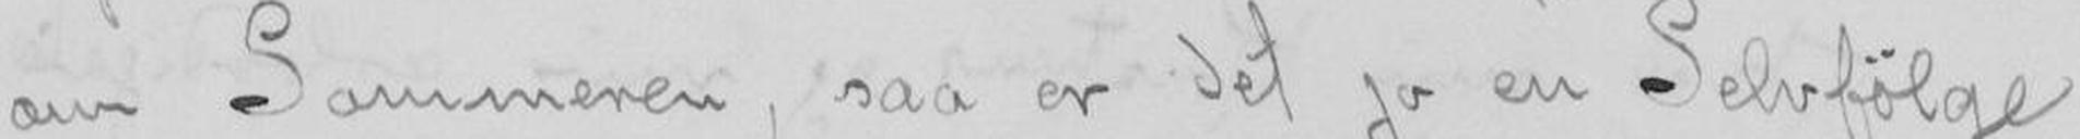om Sommeren, saa er det jo en Selvfølge
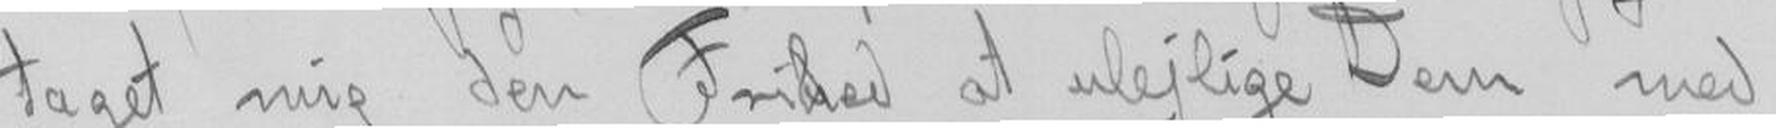taget mig den Frihed at ulejlige Dem med
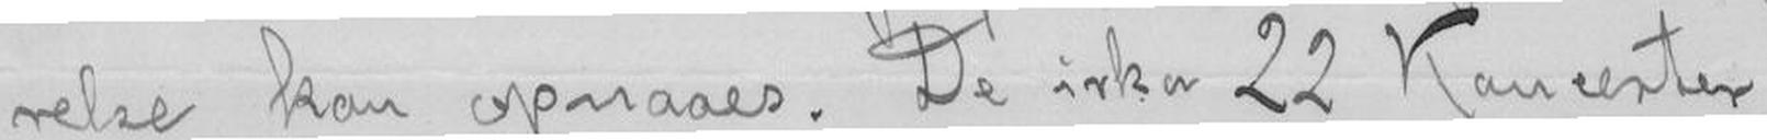relse kan opnaaes. De (c)irka 22 Koncerter
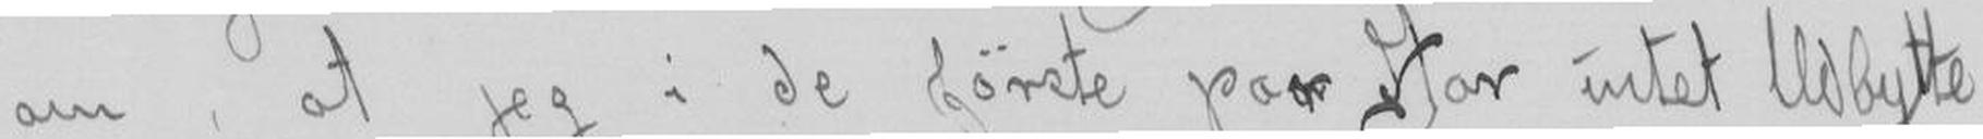om at jeg i de første par Aar intet Udbytte
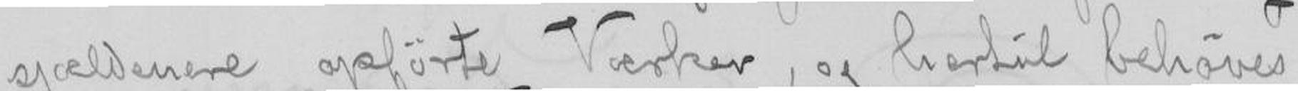sjældenere opførte Værker, og hertil behøves
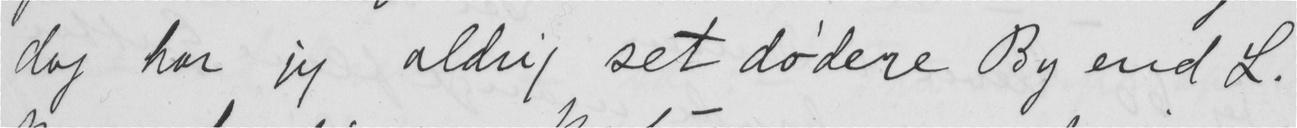dog har jeg aldrig set dødere By end L.
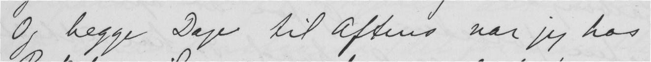Og begge Dage til Aftens var jeg hos
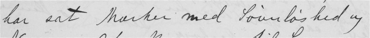har sat Mærker med Søvnløshed og
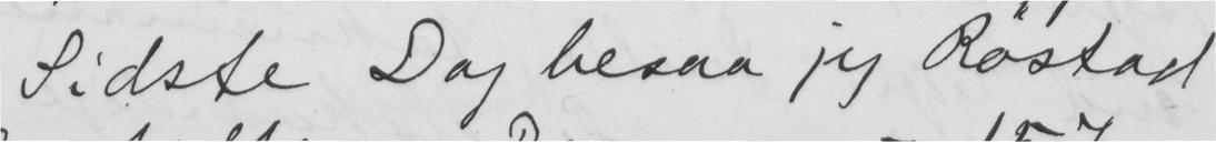Sidste Dag besaa jeg Røstad
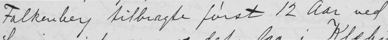Falkenberg tilbragte først 12 Aar ved
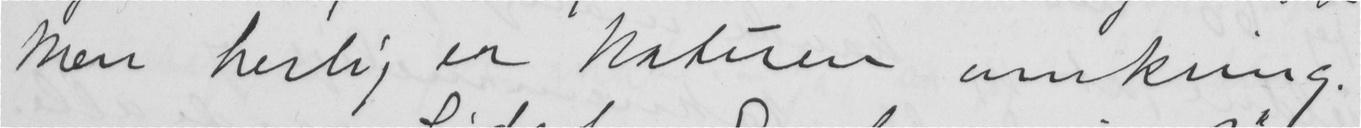Men herlig er Naturen omkring.
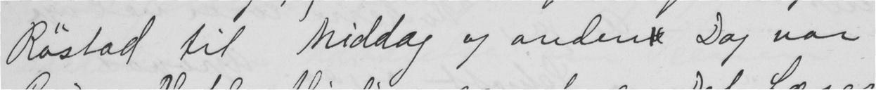Røstad til Middag og anden Dag var
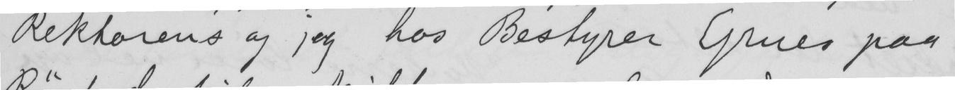Rektorens og jeg hos Bestyrer Grues paa
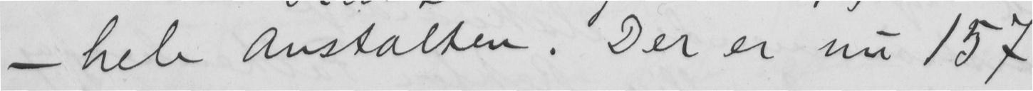- hele Anstalten. Der er nu 157
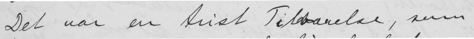Det var en trist Tilværelse, som
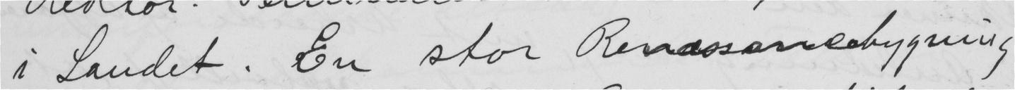i Landet. En stor Renæssancebygning
